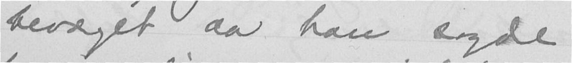bevæget da han sagde
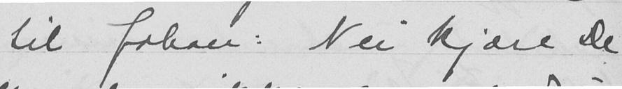til Johan: Nei kjære De
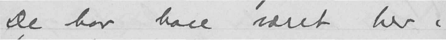De har bare været her i
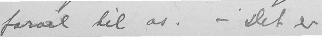farvel til os. - Det er
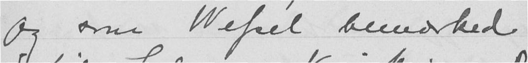Og som Wessel bemærked
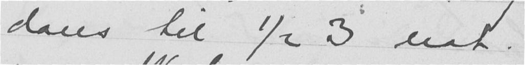dans til ½ 3 nat.
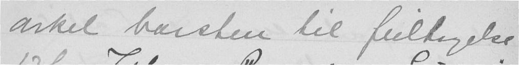onkel Carsten til feiltagelse
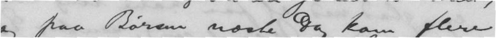og paa Børsen næste Dag kom flere
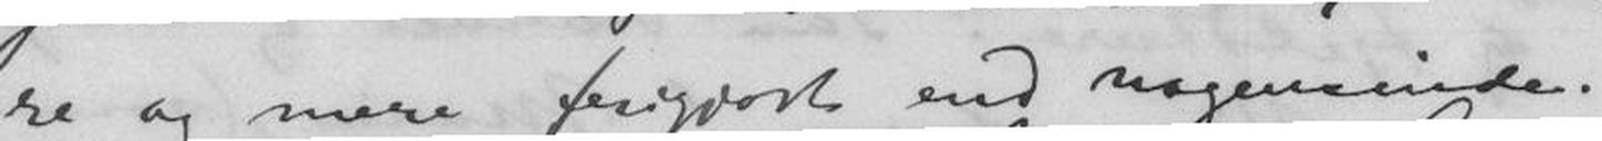re og mere frigjort end nogensinde.
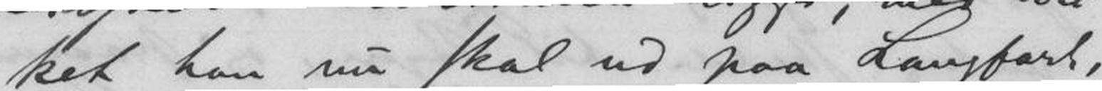ket han nu skal ud paa Langfart,
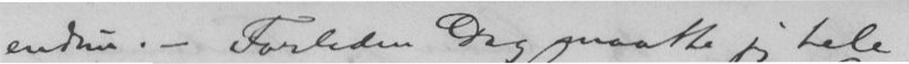endnu. - Forleden Dag maatte jeg tele-
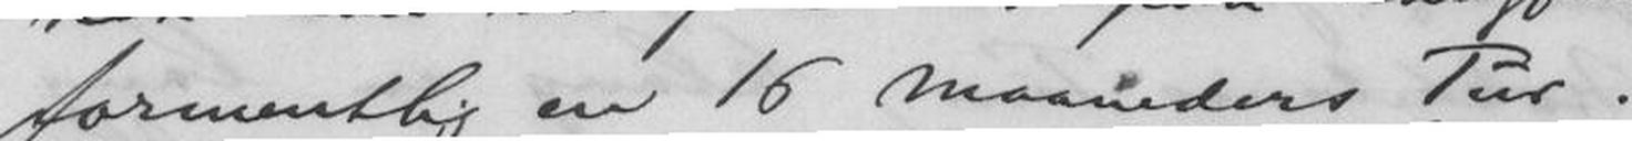formentlig en 16 Maaneders Tur.
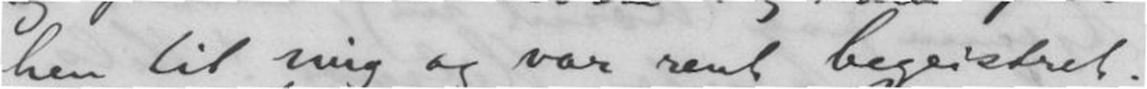hen til mig og var rent begeistret.
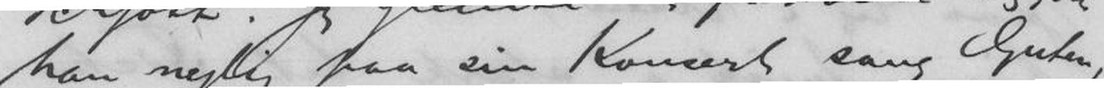han nylig paa sin Konsert sang Guten,
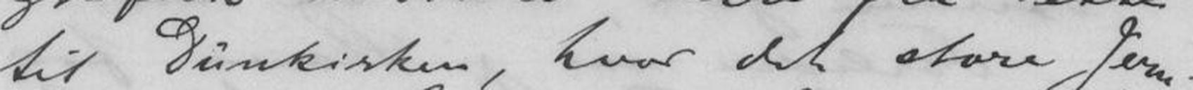til Dunkirkue, hvor det store Jern-
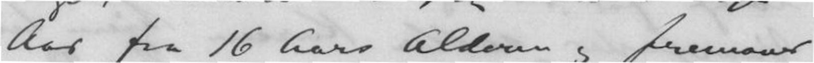Aar fra 16 Aars Alderen og fremover
